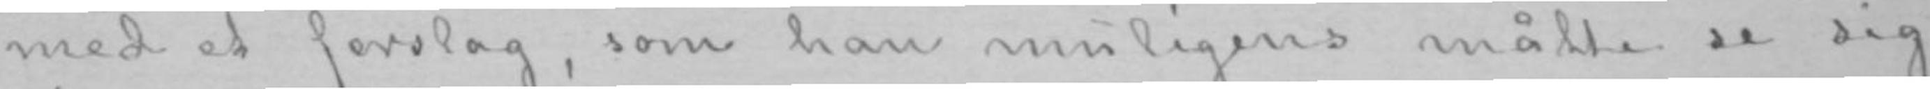med et forslag, som han muligens måtte se sig
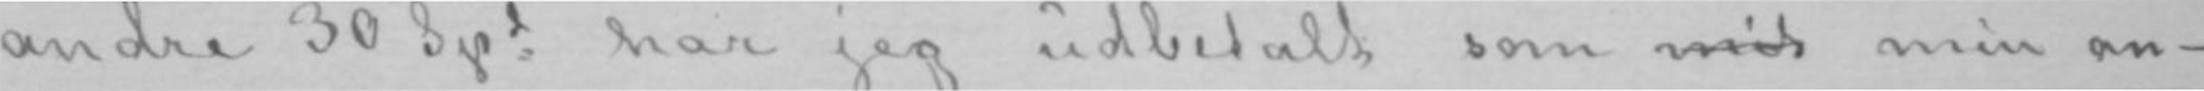andre 30 Spd har jeg udbetalt som mit min an-
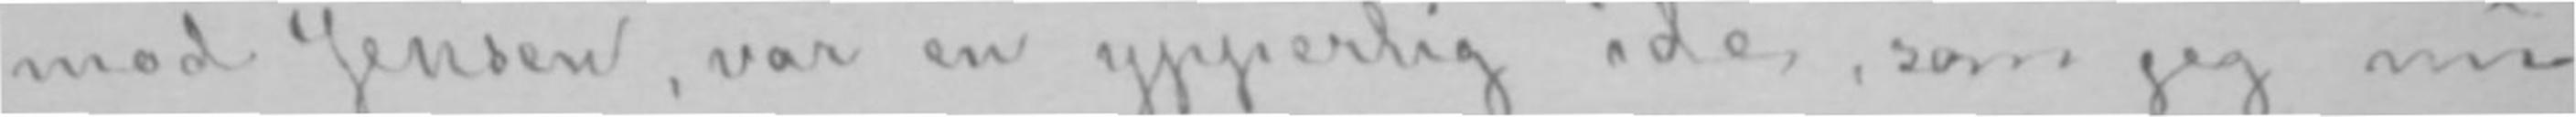mod Jensen, var en ypperlig ide, som jeg nu
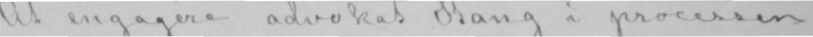At engagere advokat Stang i processen
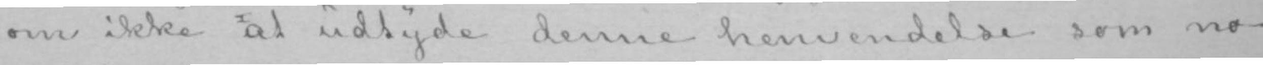om ikke uat udtyde denne henvendelse som no-
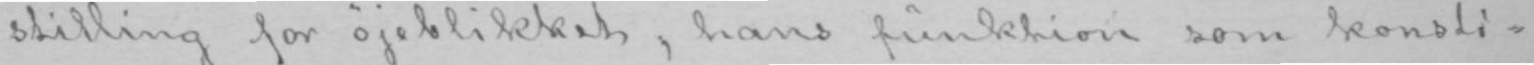stilling for øjeblikket; hans funktion som konsti-
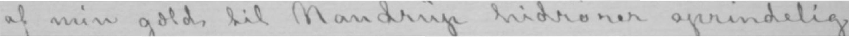af min gæld til Nandrup hidrører oprindelig
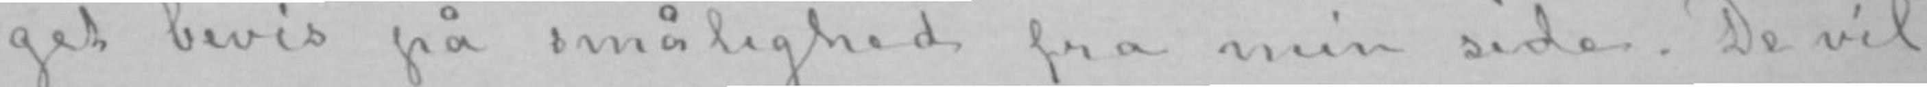get bevis på smålighed fra min side. De vil
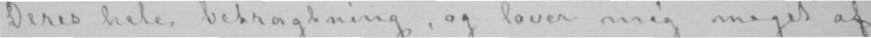Deres hele betragtning, og lover mig meget af
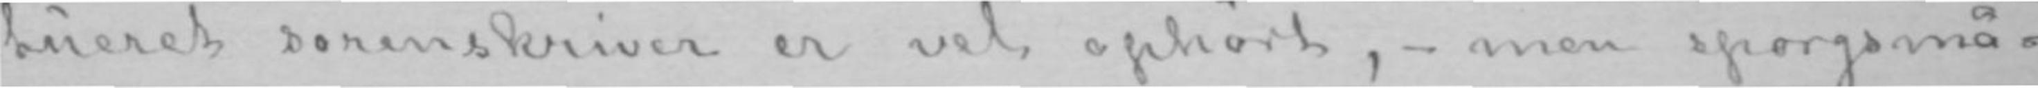tueret sorenskriver er vel ophørt,- men spørgsmå-
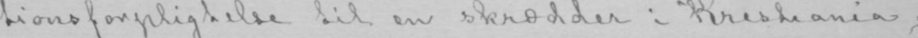tionsforpligtelse til en skrædder i Kristiania;
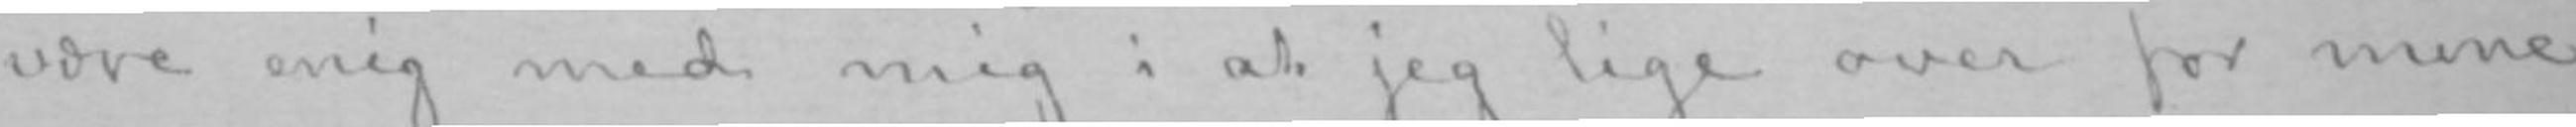være enig med mig i at jeg lige over for mine
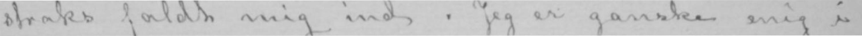straks faldt mig ind. Jeg er ganske enig i
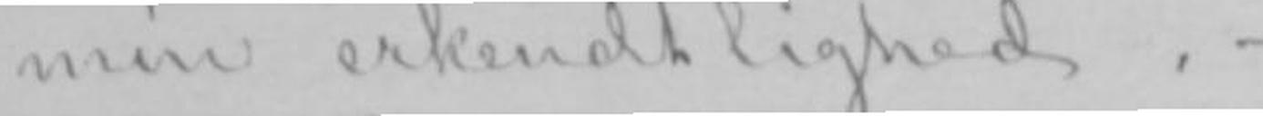min erkendtlighed.-
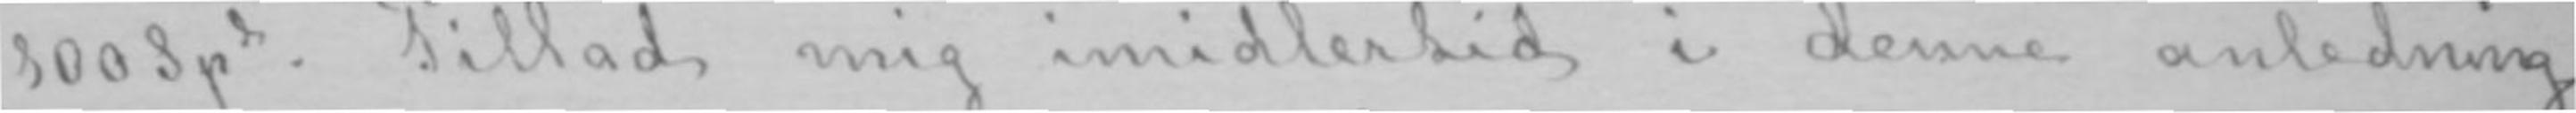100 Spd. Tillad mig imidlertid i denne anledning
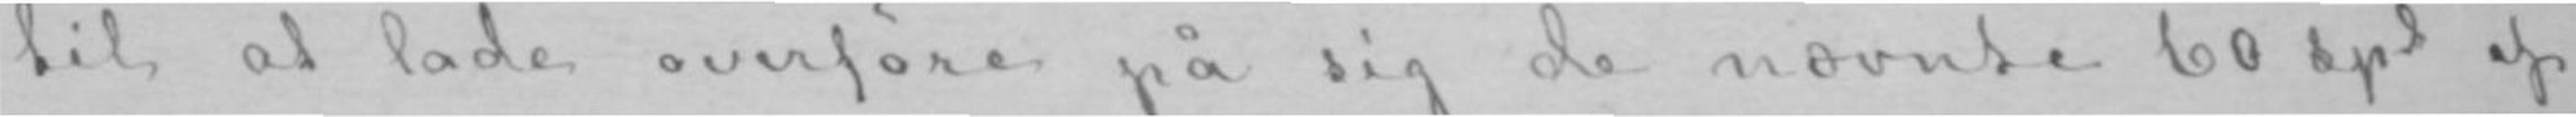til at lade overføre på sig de nævnte 60 Spd af
